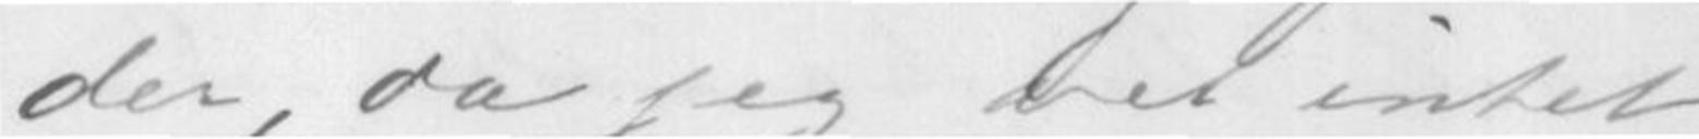der, da jeg vel intet
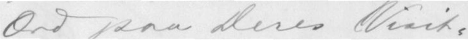Ord paa Deres Visit-
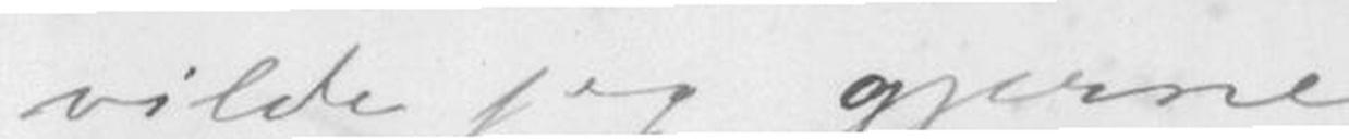vilde jeg gjerne
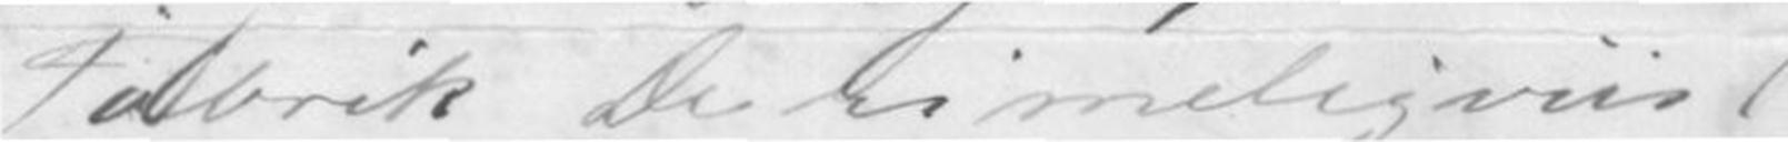Fabrik De rimeligviis
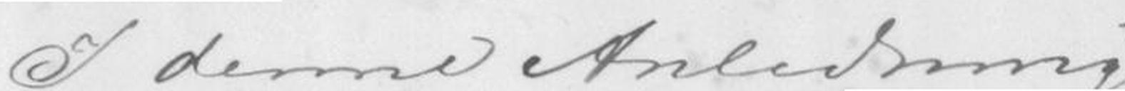I denne Anledning
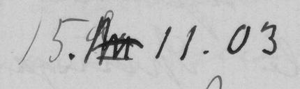15. 11.03
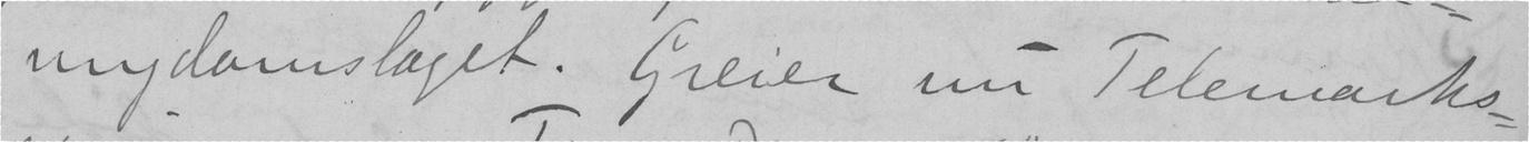ungdomslaget. Greier nu Telemarks-
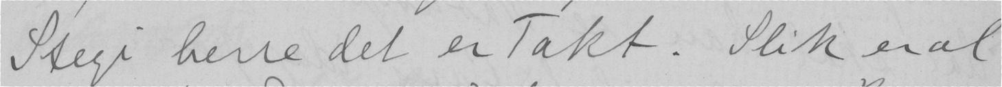Stegi herre det er Takt. Slik er al
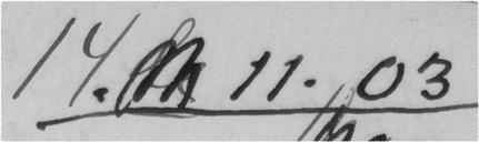14.  11.03
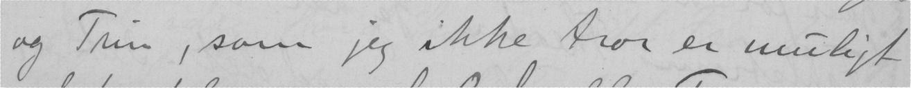og Trin, som jeg ikke tror er muligt.
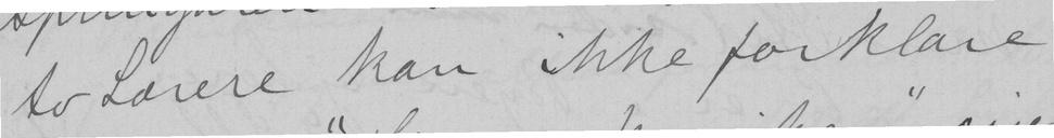to Lærere kan ikke forklare
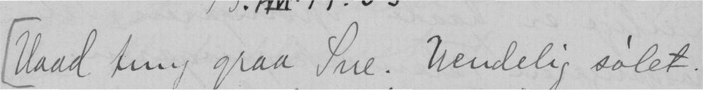Vaad tung graa Sne. Uendelig sølet.
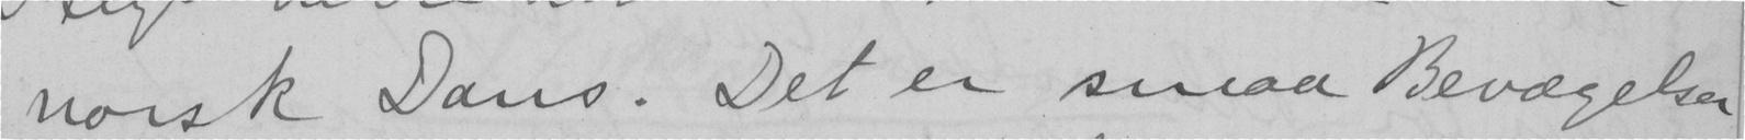norsk Dans. Det er smaa Bevægelser
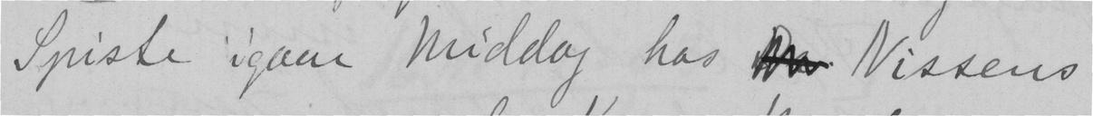Spiste igaar middag hos  Nissens
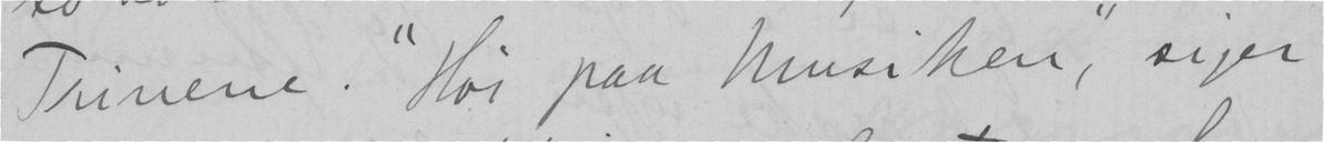Trinene. "Hør paa Musiken", siger
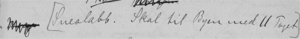Sneslabb. Skal til Byen med 11 Toget.
